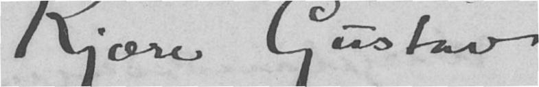Kjære Gustav
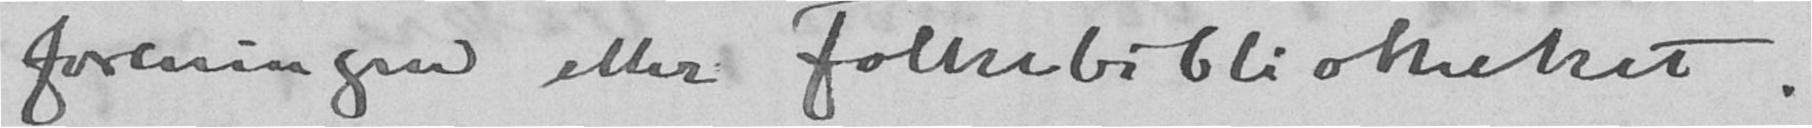foreningen eller Folkebiblioteket.
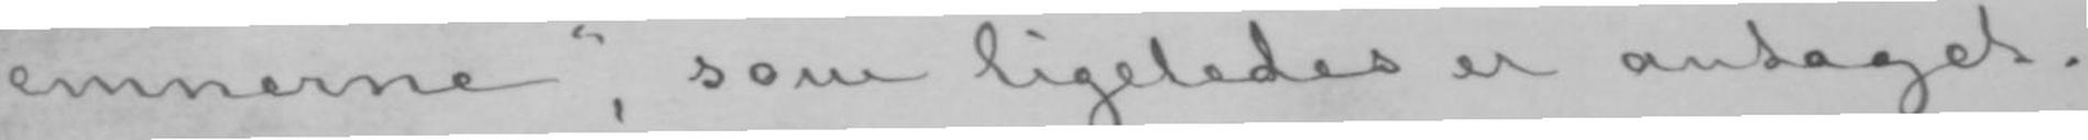emnerne», som ligeledes er antaget.
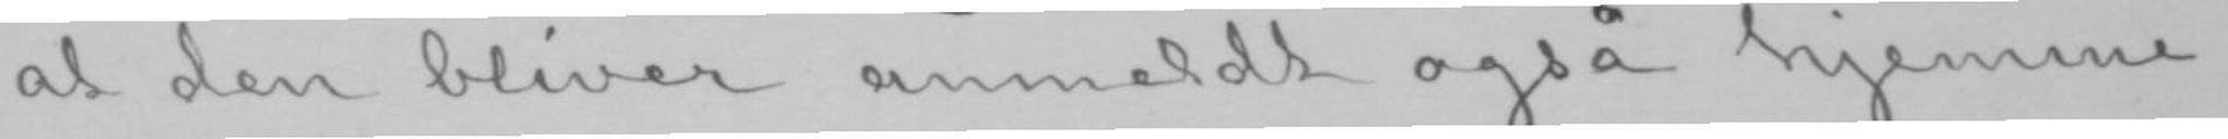at den bliver anmeldt også hjemme.
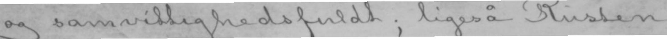og samvittighedsfuldt; ligeså Rusten
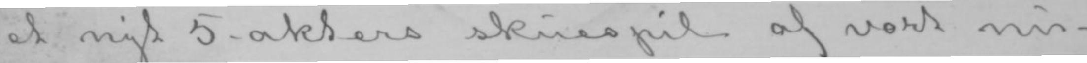et nyt 5-akters skuespil af vort nu-
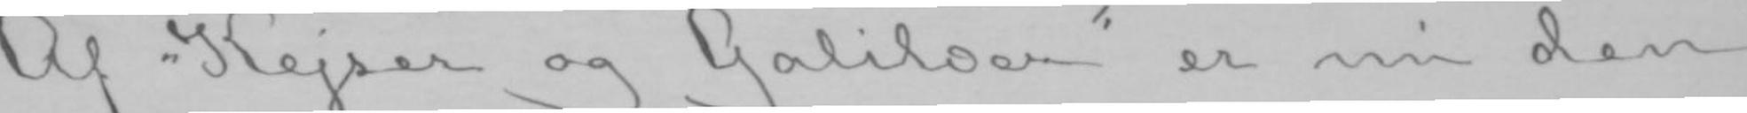Af «Kejser og Galilæer» er nu den
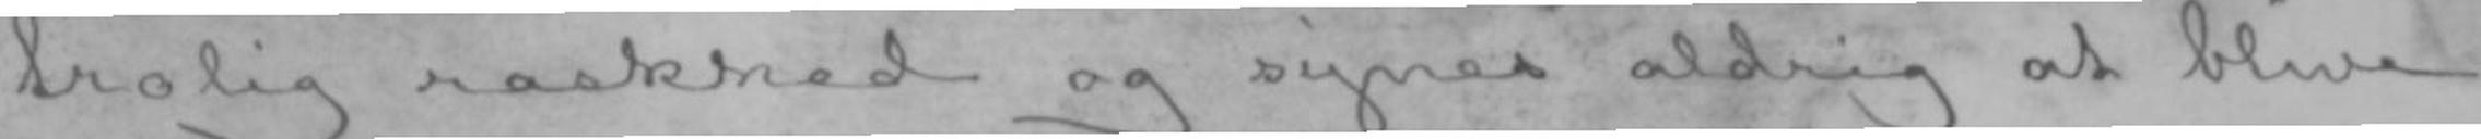trolig raskhed og synes aldrig at blive
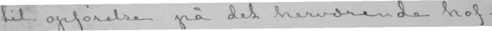til opførelse på det herværende hof-
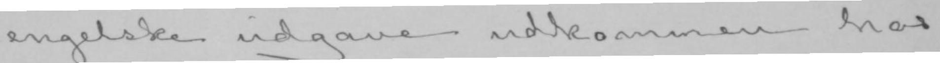engelske udgave udkommen hos
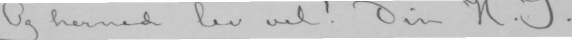Og hermed lev vel! Din H. I.
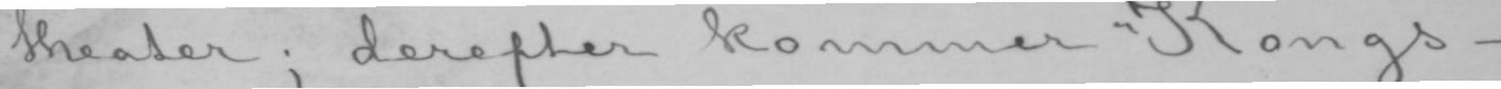theater; derefter kommer «Kongs-
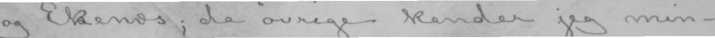og Ekenæs; de øvrige kender jeg min-
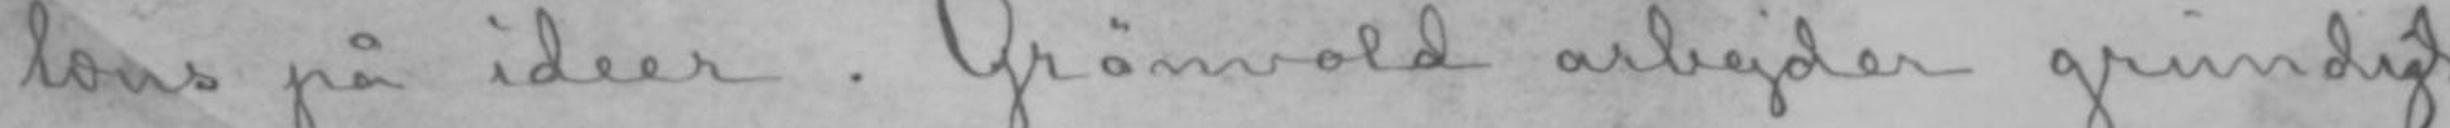læns på ideer. Grønvold arbejder grundigt
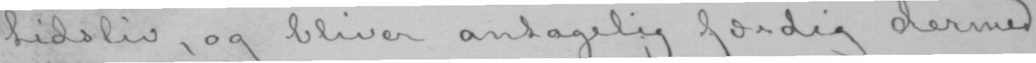tidsliv, og bliver antagelig færdig dermed
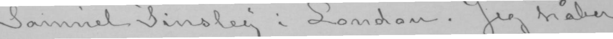Samuel Tinsley i London. Jeg håber
